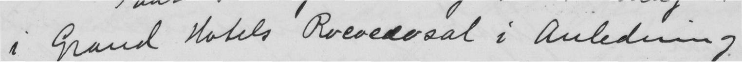i Grand Hotels Rococcosal i Anledning
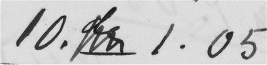10. 1.05
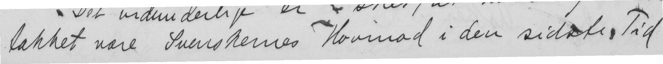takket være Svenskernes Hovmod i den sidste Tid
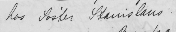hos Søster Stanislaus.
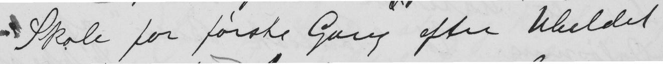Skole for første Gang efter Uheldet
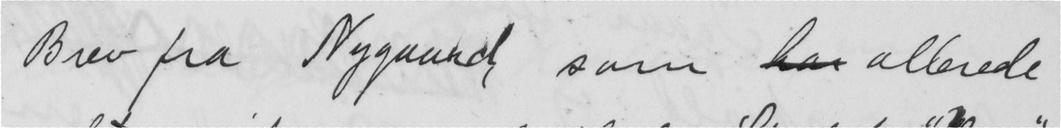Brev fra Nygaard som  allerede
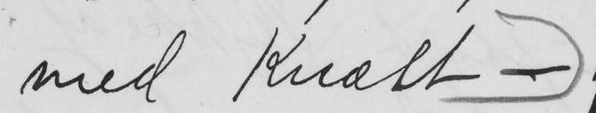med Knæet -
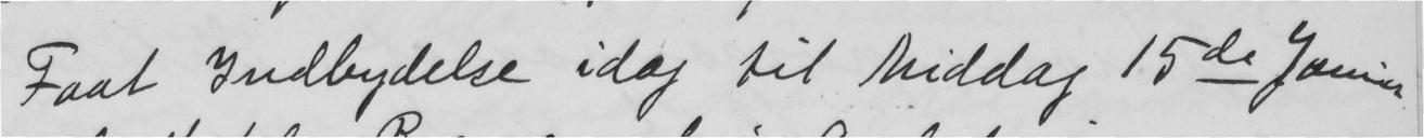Faat Indbydelse idag til Middag 15de Januar
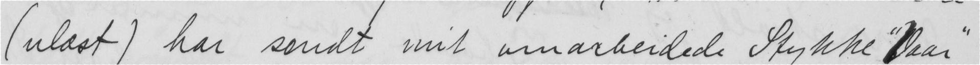(ulæst) har sendt mit omarbeidede Stykke "Vaar"
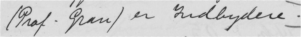(Prof. Gran) er Indbydere.
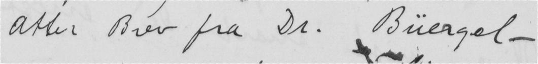Atter Brev fra Dr. Büergel -
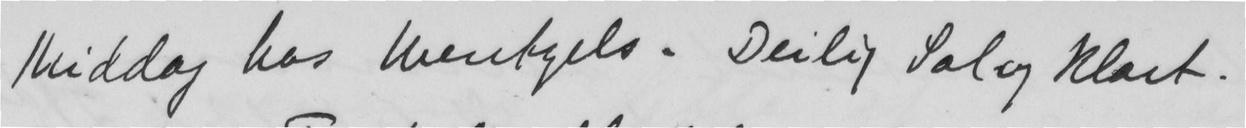Middag hos Wentzels. Deilig Sol og Klart.
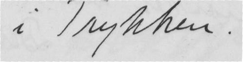i Trykken
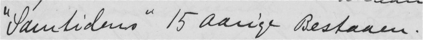"Samtidens" 15 Aarige Bestaaen.
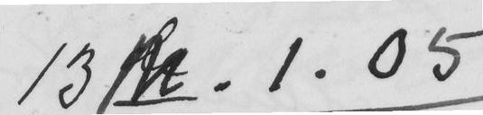13. 1.05
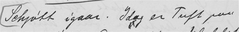Schjøtt igaar. Idag er Tuft paa
